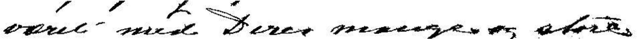været med Deres mange og store
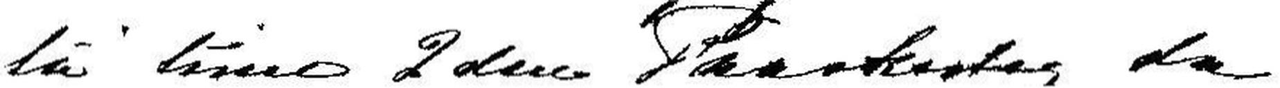ta time 2 den Paaskedag da
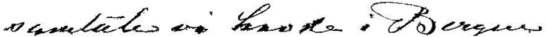samtale vi havde i Bergen
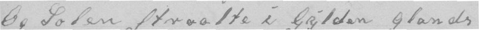Og Solen straalte i Gylden glands
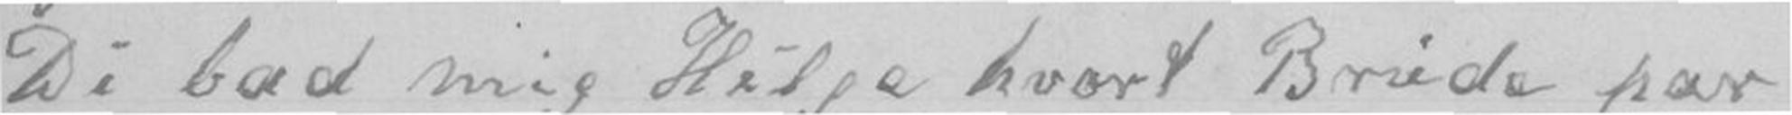Di bad mig Hilse hvort Brude par
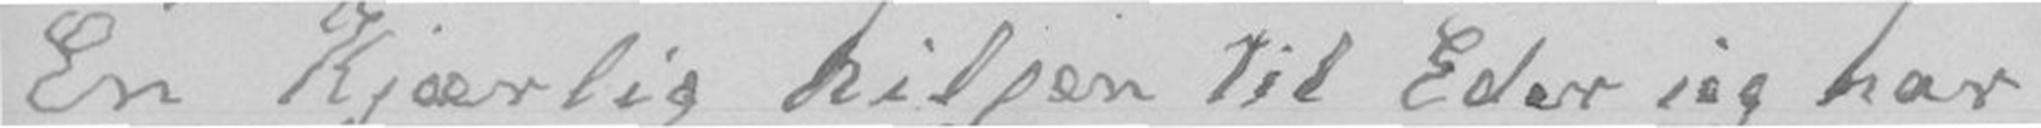En Kjærlig hilsen til Eder ieg har
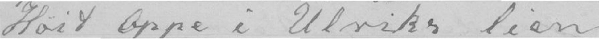Høit Oppe i Ulriks lien
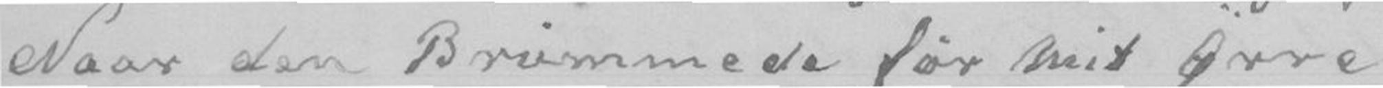Naar den Brummede før mit Ørre
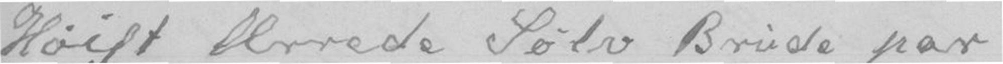Høist Ærrede Sølv Brude par
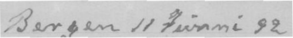Bergen 11 Junni 92
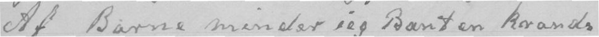Af Barne minder ieg Bant krands
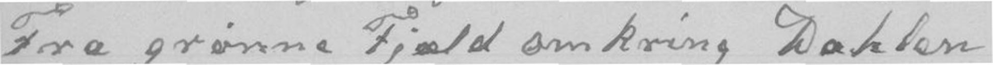Fra grønne Fjæld omkring Dahlen
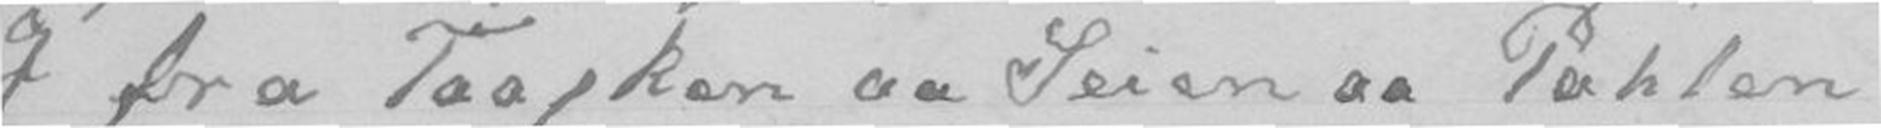I fra Taasken aa Seien aa Pahlen
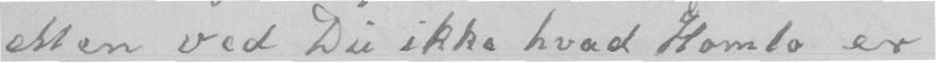Men ved Du ikke hvad Homlo er
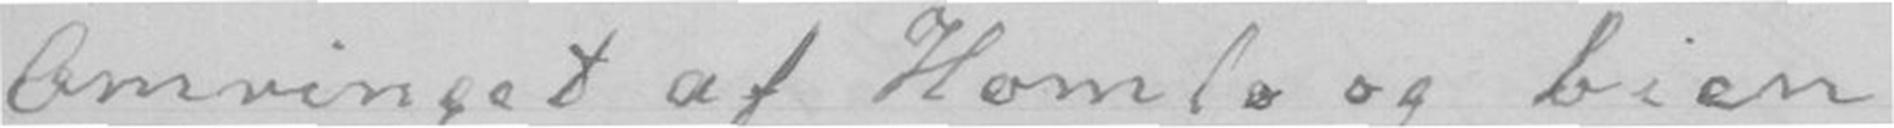Omringet af Homlo og bien
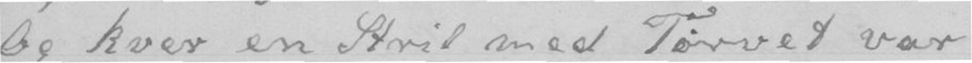Og kver en Stril med Tørvet var
